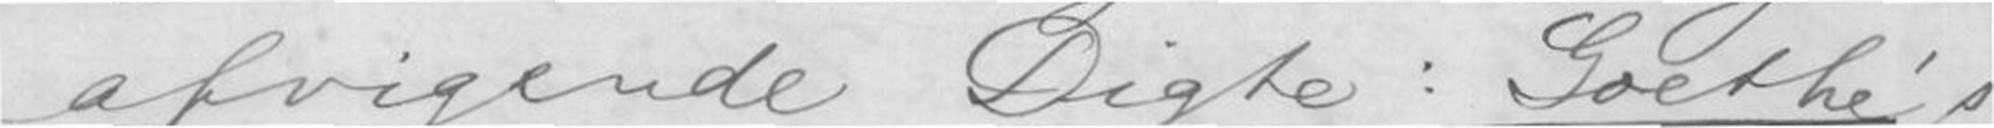afvigende Digte: Goethe's
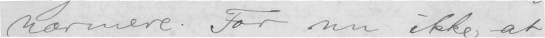nærmere. For nu ikke at
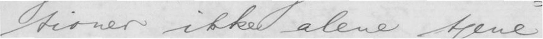sioner ikke alene tjene
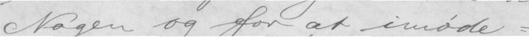Nogen og for at imøde-
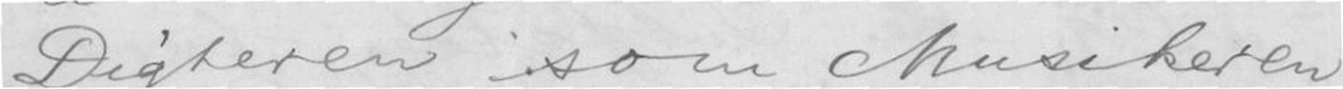Digteren som Musikeren
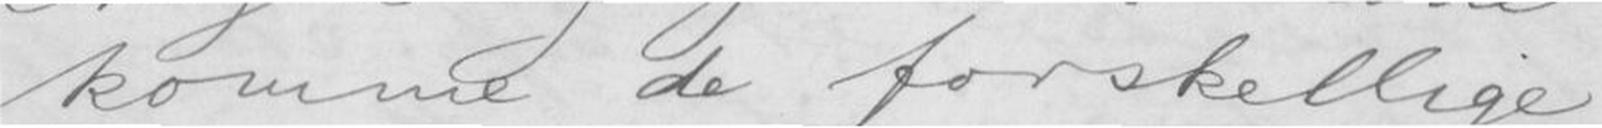komme de forskellige
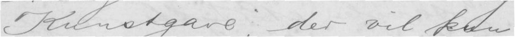Kunstgave, der vil kun
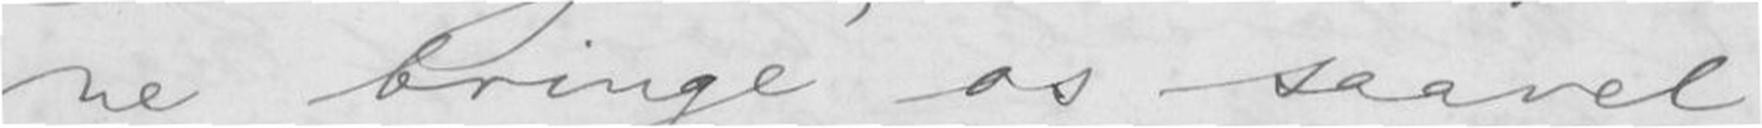ne bringe os saavel
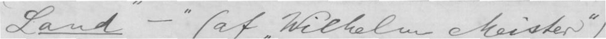Land- "(af Wilhelm Meister")
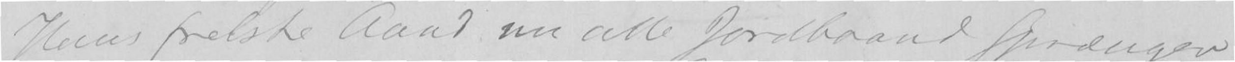Hans frelste Aand nu alle Jordbaand sprænger
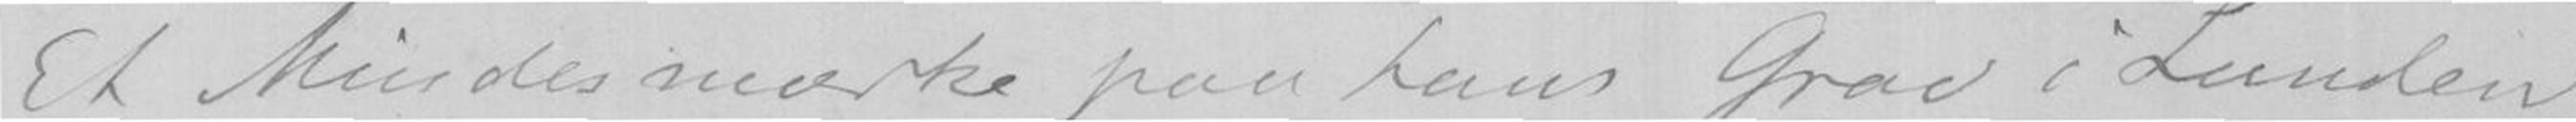Et Mindesmærke paa hans Grav i Lunden
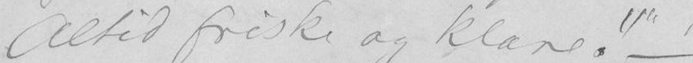Altid friske og klare. -
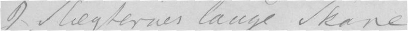I Slegternes lange Skare
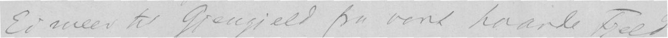Ei meer til Gjengjeld fra vort haarde Fjeld
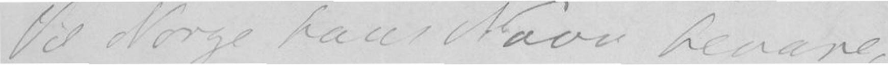Vil Norge hans Navn bevare,
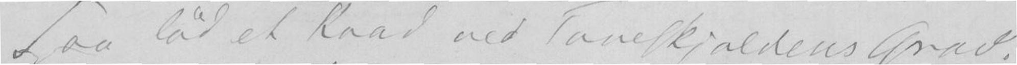Saa lød et Kvad ved Toneskjoldens Grav.
